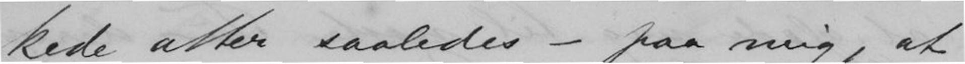kede atter saaledes - paa mig,
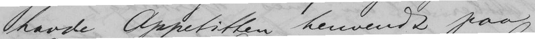havde Appetitten henvendt paa
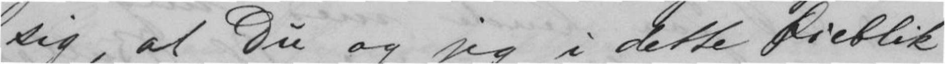sig, at Du og jeg i dette Øieblik
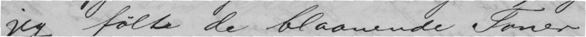jeg følte de blaanende Toner
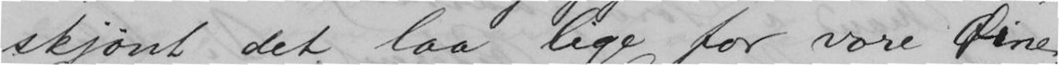skjønt det laa lige for vore Øine.
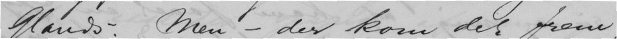Glands. Men - der kom det frem,
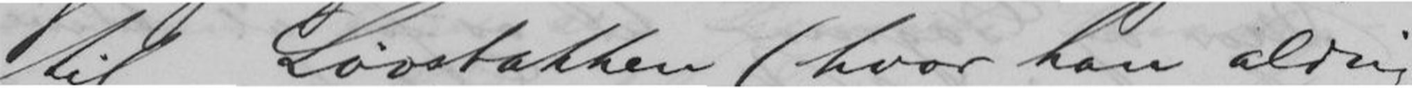til Løvstakken (hvor han aldrig
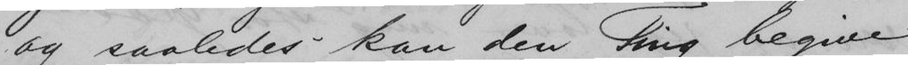og saaledes kan den Ting begive
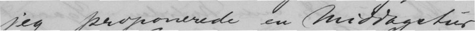at jeg proponerede en Middagstur
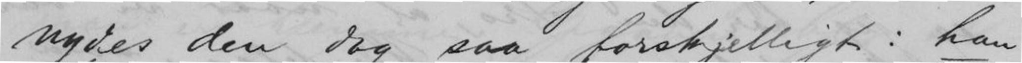nydes den dog saa forskjelligt: han
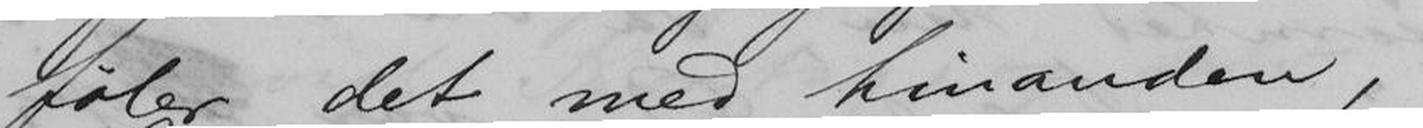føler det med hinanden,
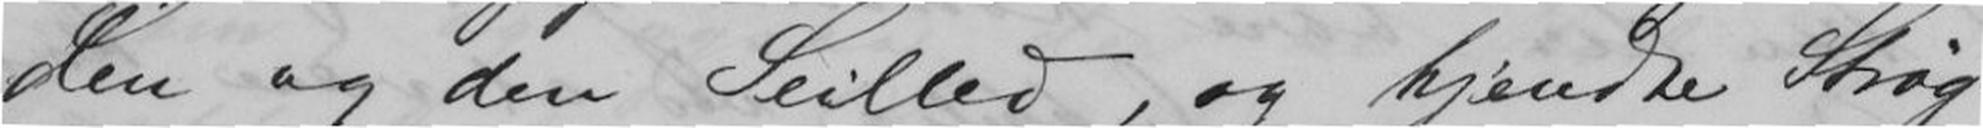den og den Seilled, og kjendte Strøg
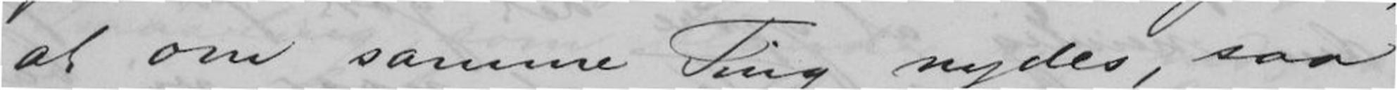at om samme Ting nydes, saa
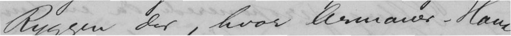Ryggen der, hvor Armauer-Hansen
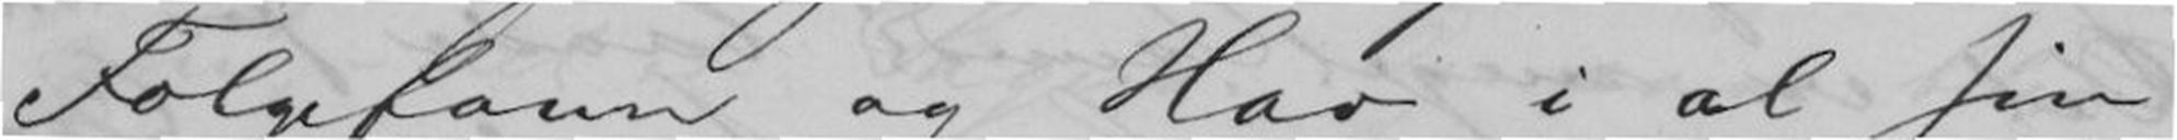Folgefonn og Hav i al sin
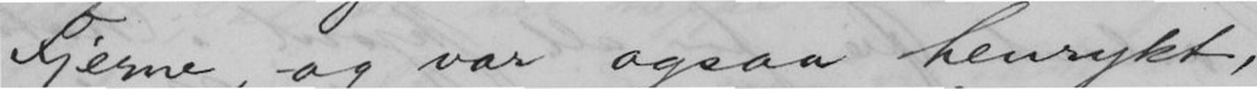Fjerne, og var ogsaa henrykt, -
